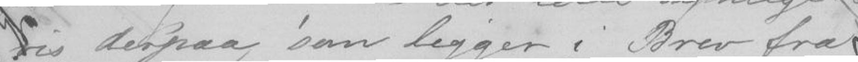vis derpaa som ligger i Brev fra en
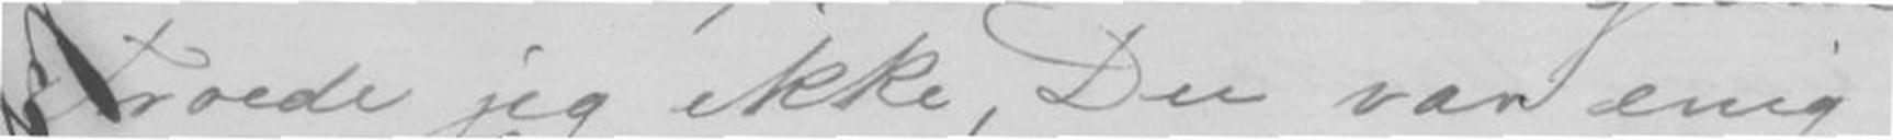Troede jeg ikke, Du var enig med
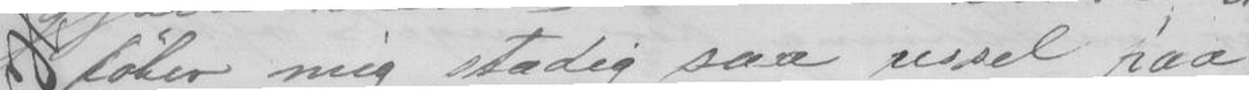føler mig stadig saa ussel paa man-
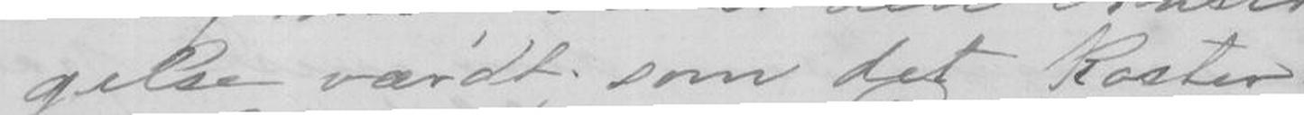gelse værdt som det koster
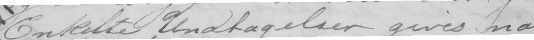Eneklte Undtagelser gives na
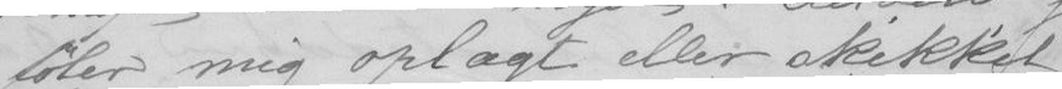føler mig oplagt eller skikket
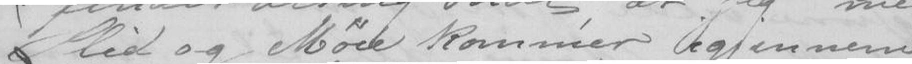Flid og Møie kommer igjennem
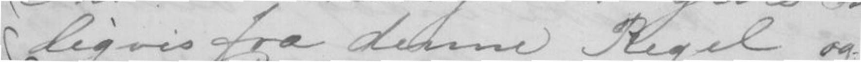ligvis fra denne Regel og d
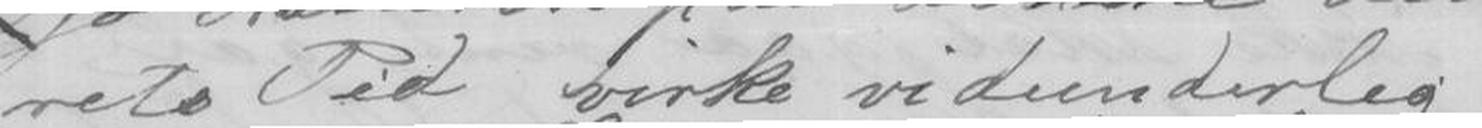rets Tid virke vidunderleg
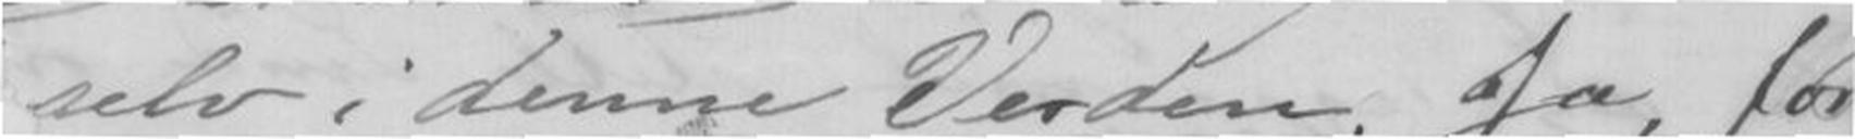selv i denne Verden. Ja, fo
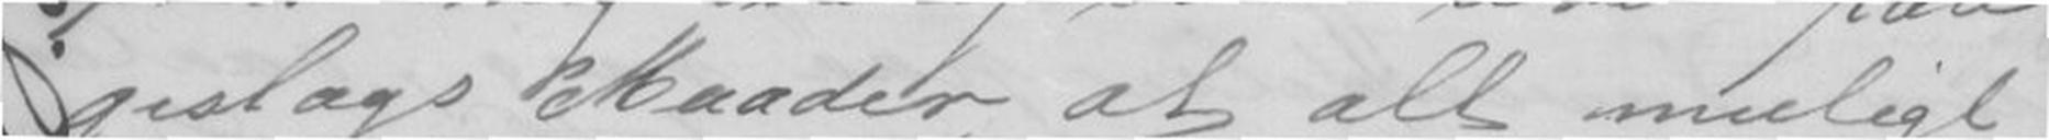geslags Maader, at alt muligt blir
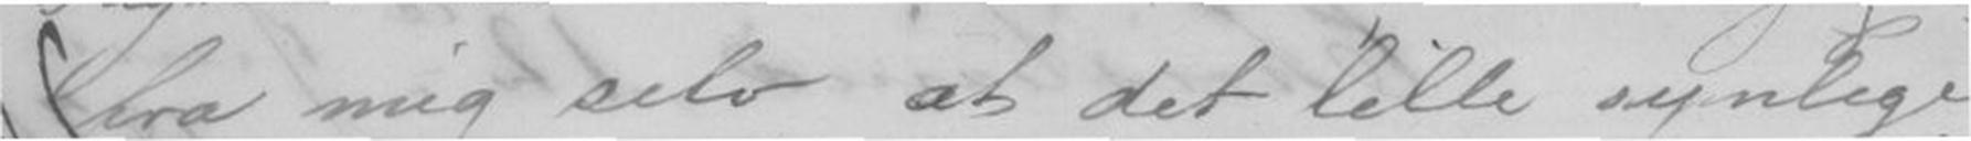fra mig selv, at det lille synlige Be-
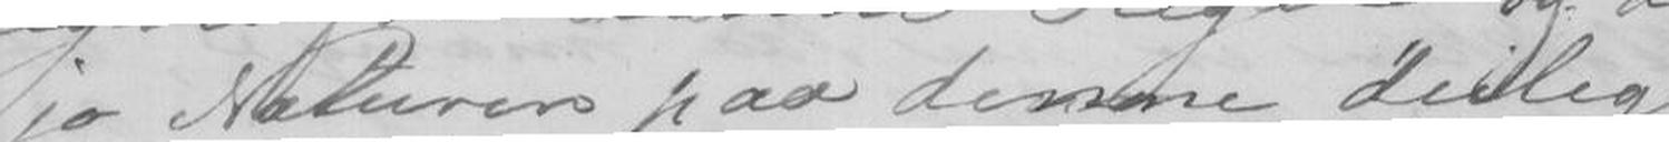jo Naturen paa denne deilig
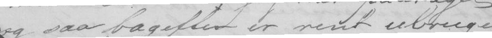og saa bagefter er rent ubruge
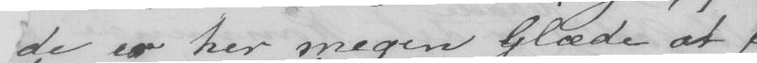de er her megen Glæde at
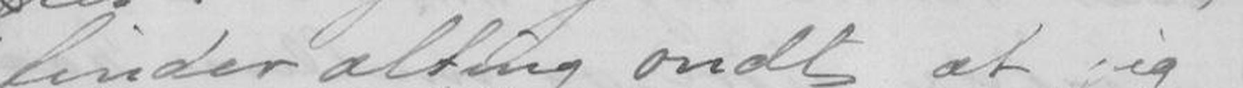finder alting ondt, at jeg
